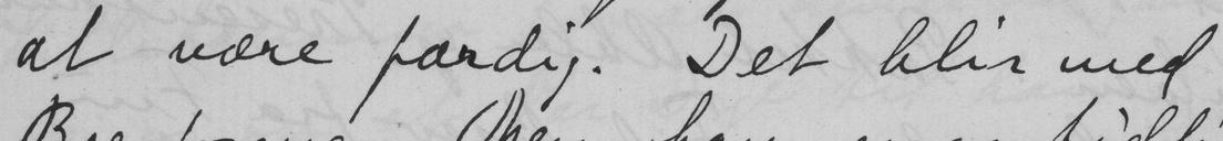at være færdig. Det blir med
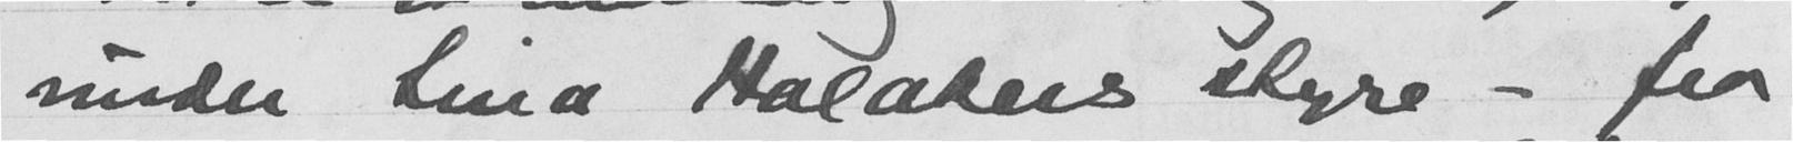under Lina Holakers styre - fra
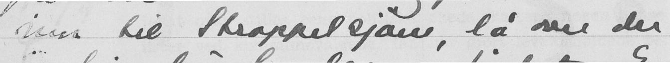inn til Strappelsjøen, lå over der
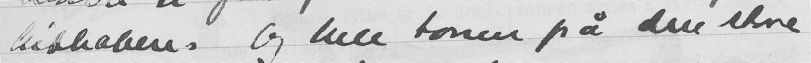Nibhabere. Og hele tonen på den store
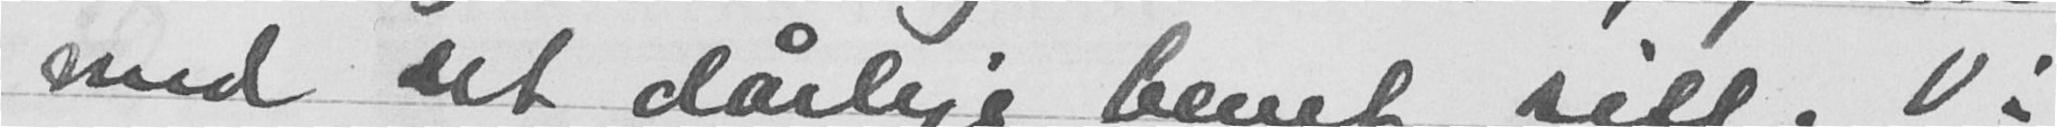med det dårlige benet sitt. Vi
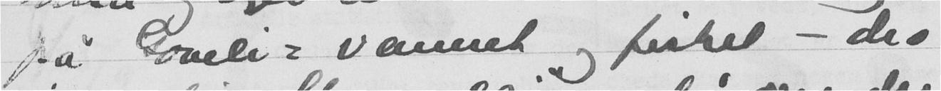på Graneli-vannet og fisket - dro
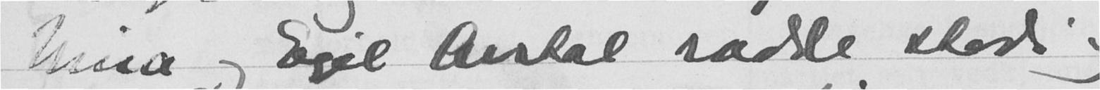Nina og Egil Arstal rodde forbi og
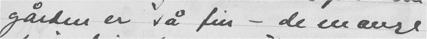gården er så fin - de mange
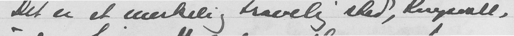Det er et merkelig og trivelig sted, Kongsvoll,
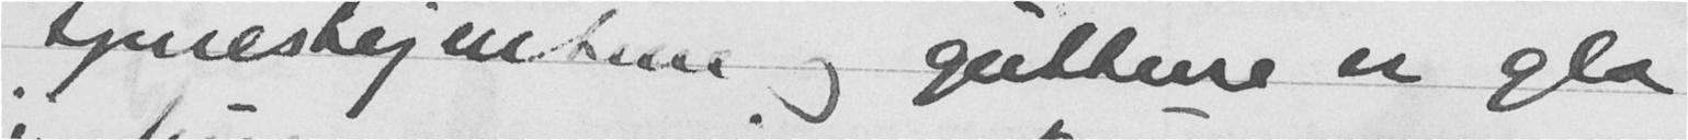tjenestejentene og guttene er gla
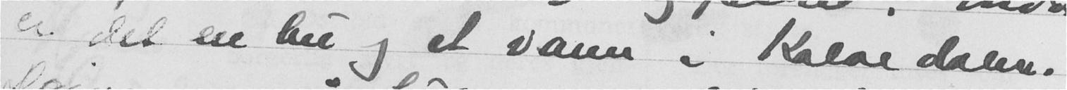er det en hei og et vann i Kalve dalen.
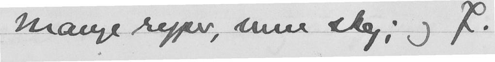Mange ryper, men skog; og J.
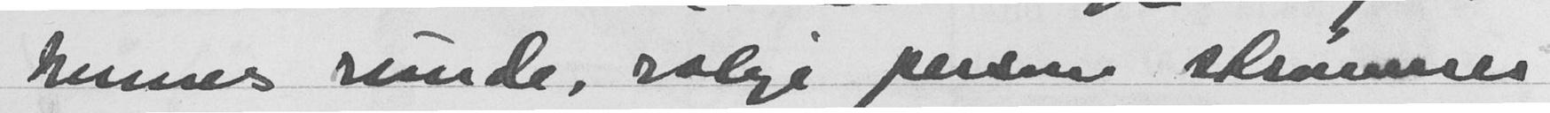hennes runde, rolige person strømmer
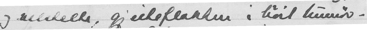og velstelte, gjeiteflokken i høit humør -
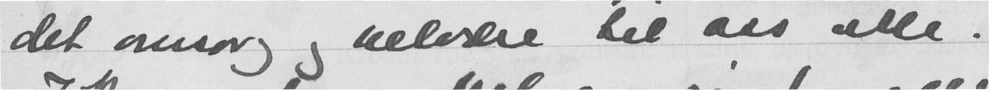det omsorg og velvære til oss alle.
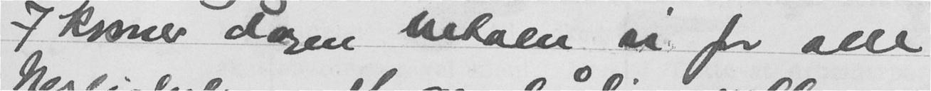7 kroner dagen betaler vi for alle
